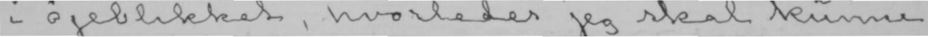i øjeblikket, hvorledes jeg skal kunne
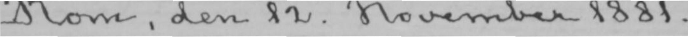Rom, den 12. November 1881.
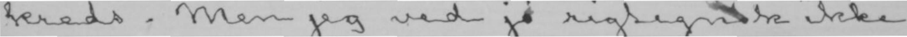kreds. Men jeg ved jo rigtignok ikke
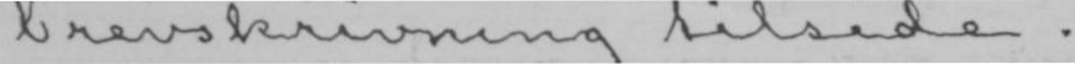brevskrivning tilside.
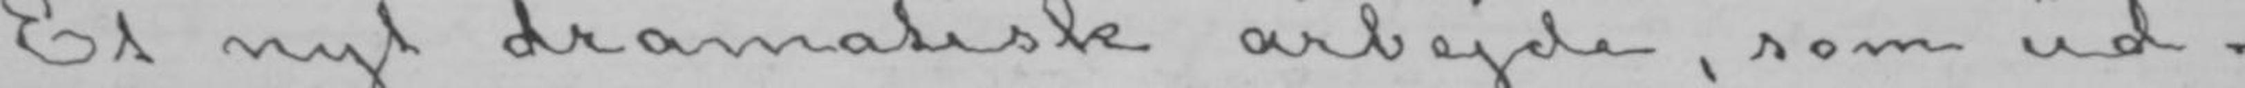Et nyt dramatisk arbejde, som ud-
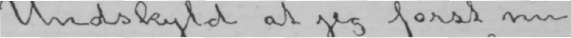Undskyld at jeg først nu
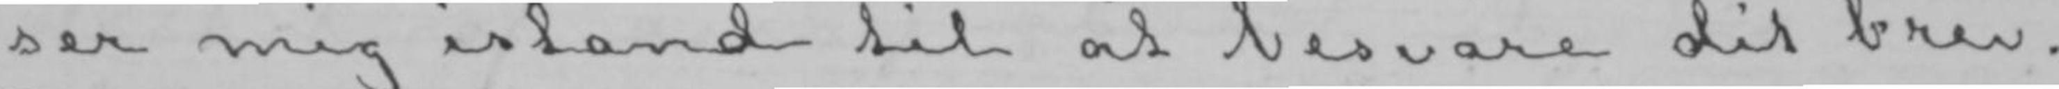ser mig istand til at besvare dit brev.
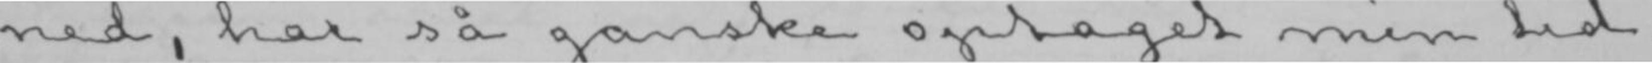ned, har så ganske optaget min tid,
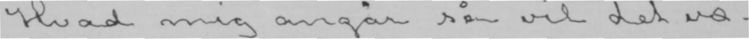Hvad mig angår så vil det væ-
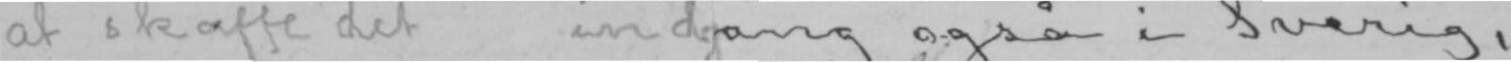at skaffe det indang også i Sverig,
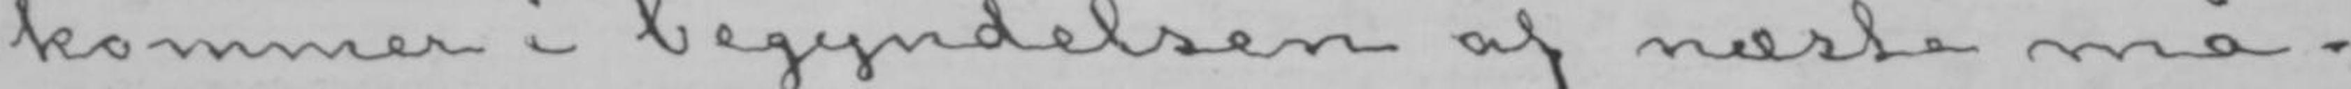kommer i begyndelsen af næste må-
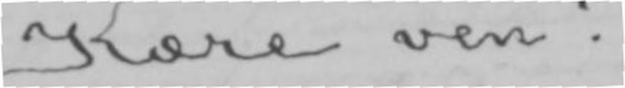Kære ven!
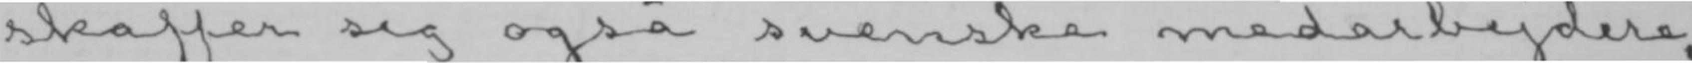skaffer sig også svenske medarbejdere,
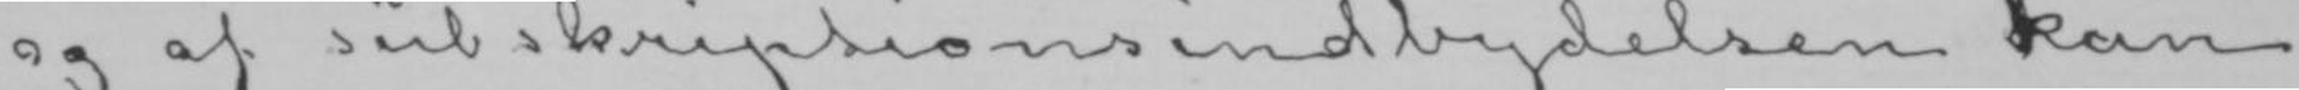og af subskriptionsindbydelsen kan
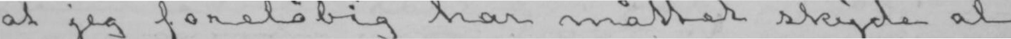at jeg foreløbig har måttet skyde al
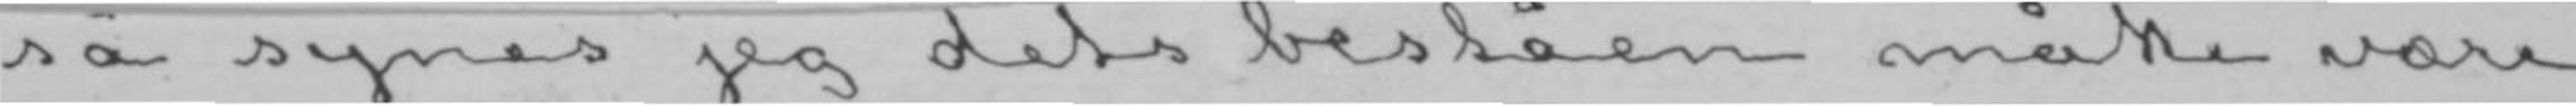så synes jeg dets beståen måtte være
